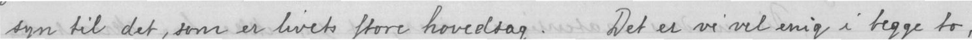syn til det, som er livets store hovedsag. Det er vi vel enige i begge to,
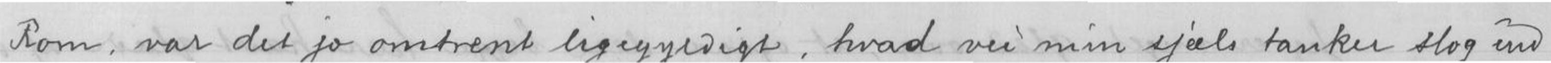Rom, var det jo omtrent ligegyldigt hvad vei min sjæls tanker slog ind
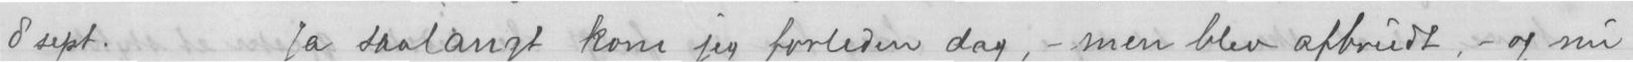8 sept. Ja saalangt kom jeg forleden dag, - men blev afbrudt, - og nu
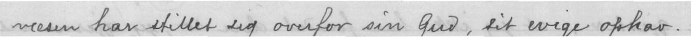væsen har stillet sig overfor sin Gud, sit evige ophav.
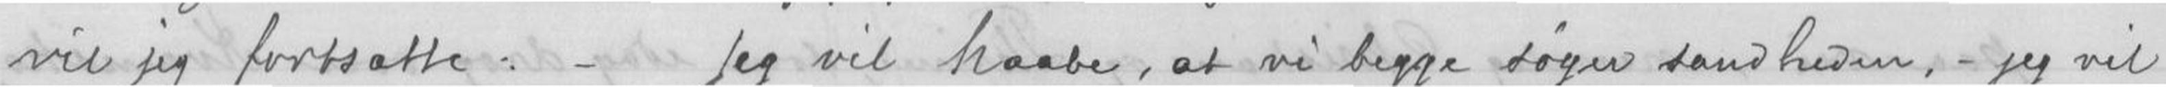vil jeg fortsætte. - Jeg vil haabe, at vi begge søger sandheden, - jeg vil
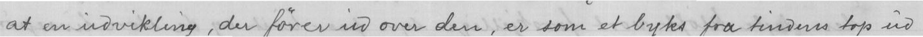at vi udvikler, der fører ud over den, er som et byks fra tinders top ud
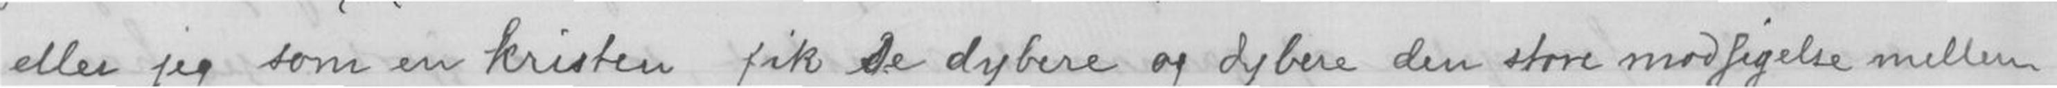eller jeg som en kristen fik se dybere den store modsigelse mellem
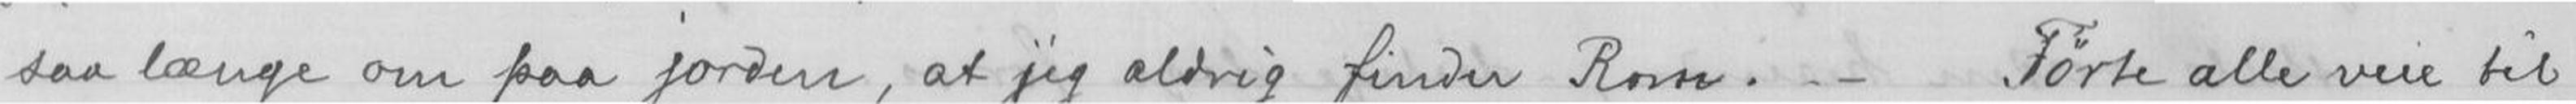saa længe paa jorden, at jeg aldrig finder Rom. - - Førte alle veie til
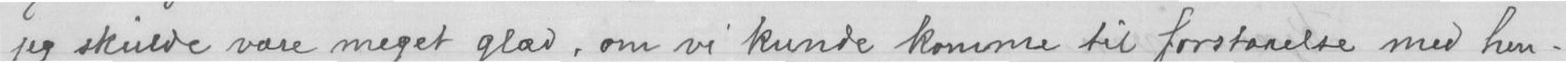jeg skulde vær meget glad, om vi kunde komme til forstaaelse med hen-
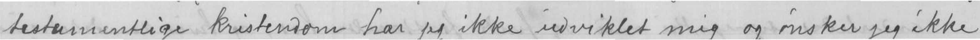testamentlige kristendom har jeg ikke udviklet mig og ønsker jeg ikke
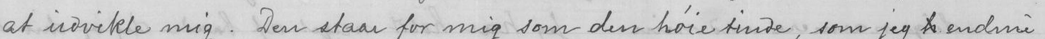at udvikle mig. Den staar for mig som den høie tinde, som jeg endnu
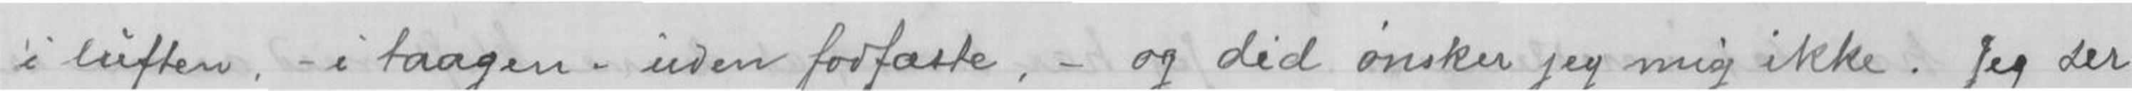i luften, - i taagen uden fodfeste, - og did ønsker jeg mig ikke. Jeg der
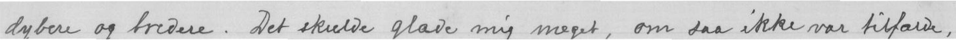dybere og bredere. Det skulde glæde mig meget, om saa ikke var tilfælde,
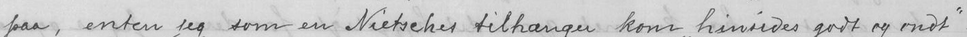paa, enten jeg som en Nietsches tilhenger kom hinsides godt og ondt -
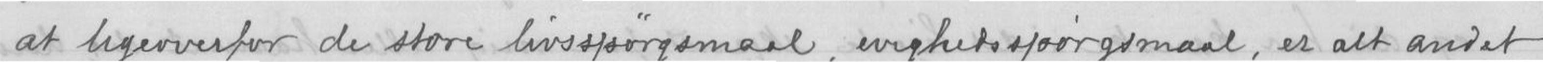at ligeoverfor de store livsspørgsmaal, evighedsspørgsmaal, er alt andet
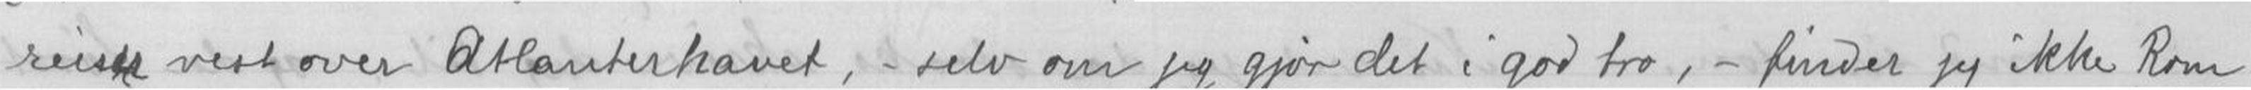reiste vest over Atlanterhavet, - selv om jeg gjør det i god tro, - finder jeg ikke Rom
